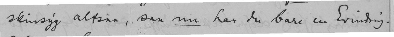skinsyg altsaa, saa nu har du bare en Erindring.
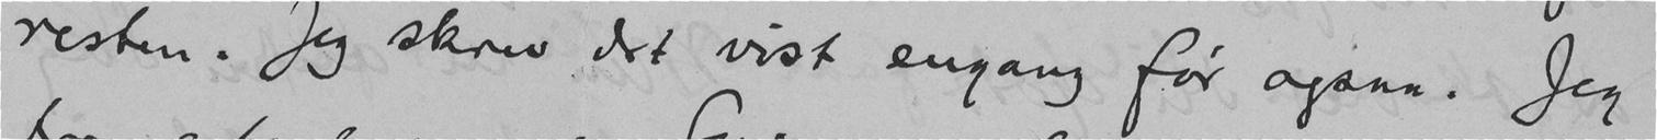resten. Jeg skrev det vist engang før ogsaa. Jeg
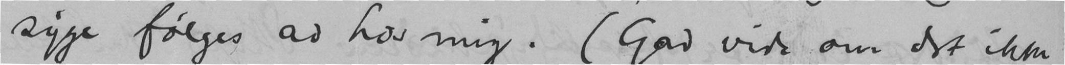syge følges ad hos mig. (Gad vide om det ikke
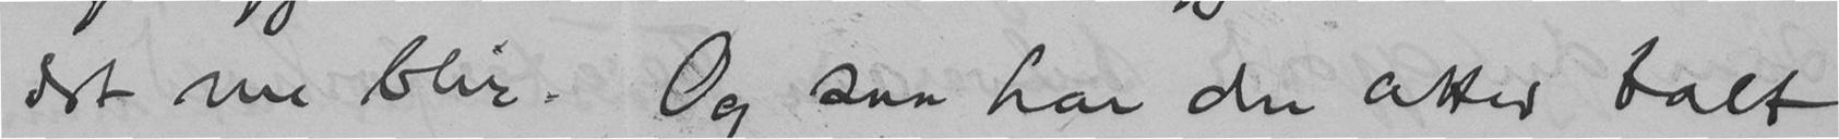det nu blir. Og saa har du atter talt
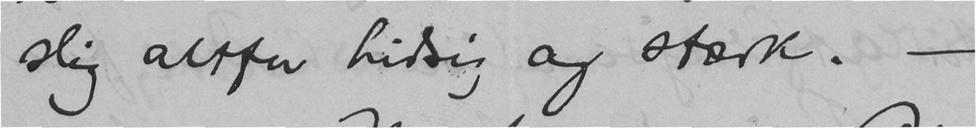slig altfor hidsig og stærk. -
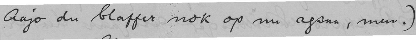Aajo du blaffer nok op nu ogsaa, men.)
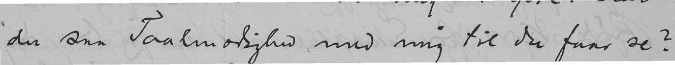du saa Taalmodighed med mig til du faar se?
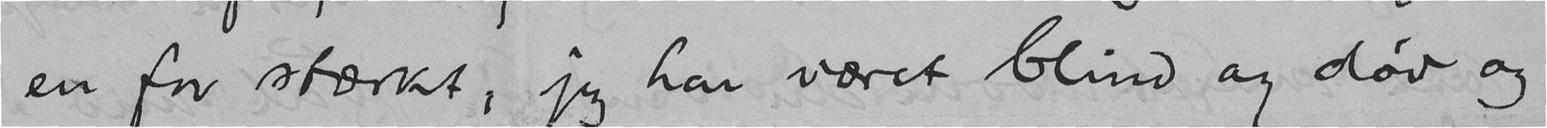en for stærkt, jeg har været blind og døv og
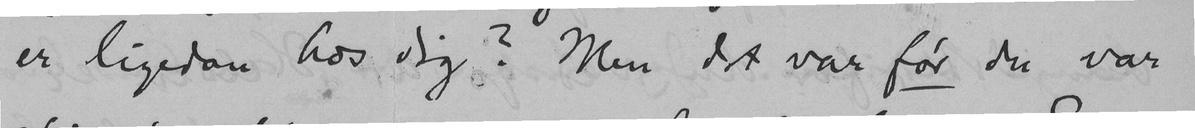er ligedan hos dig? Men det var før du var
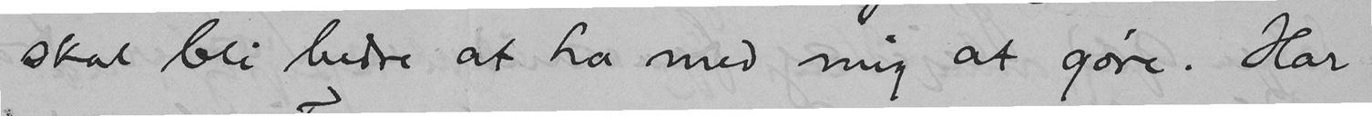skal bli bedre at ha med mig at gøre. Har
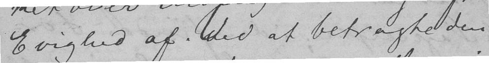Evighed af. Ved at betragte den
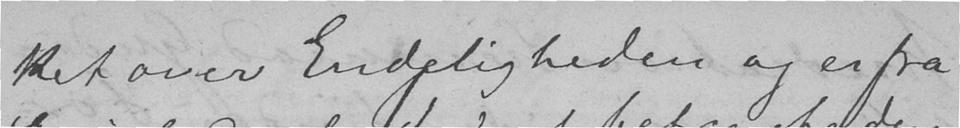ket over Endeligheden og er fra
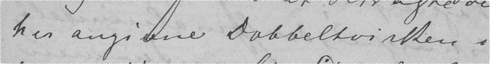her angivne Dobbeltvirken i
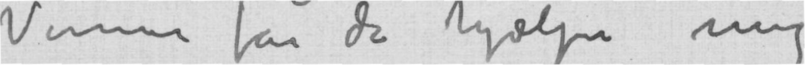Venner får da hjælpe mig
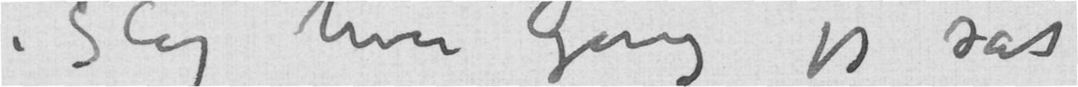i Slag hver Gang jeg sat
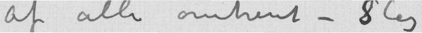af alle omtrent – Slag
